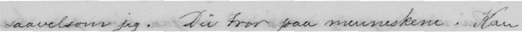saavelsom jeg. Du tror paa menneskene. Kan
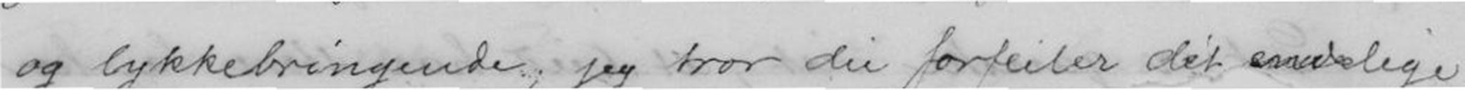og lykkebringende; jeg tror du forfeiler det endelige
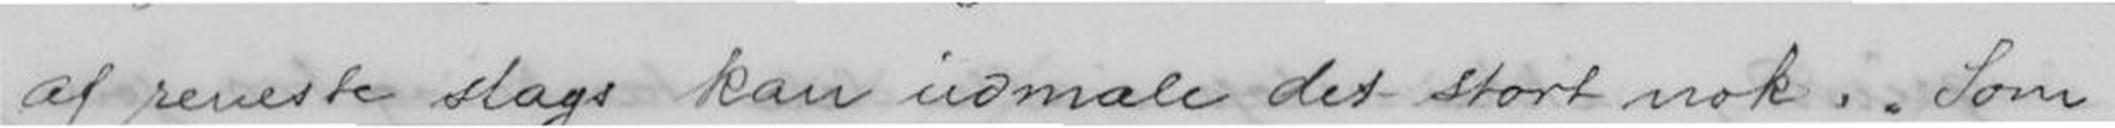af reneste slags kan udmale det stort nok. Som
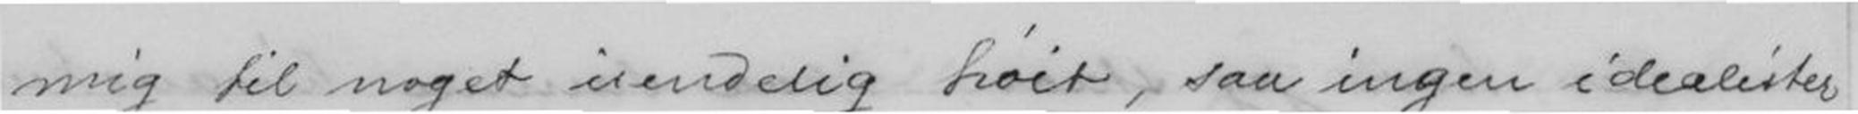mig til noget uendelig høit, saa ingen idealister
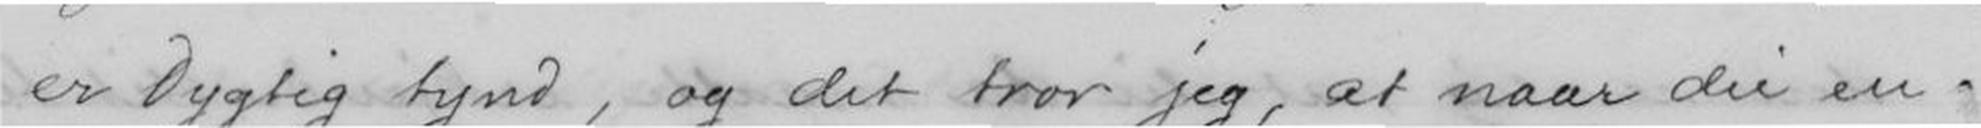er Dygtig tynd, og det tror jeg, at naar du en-
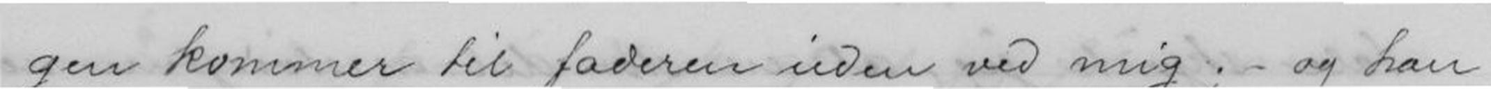gen kommer til faderen uden ved mig; - og han
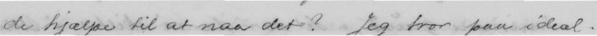de hjælpe til at naa det? Jeg tror paa ideal-
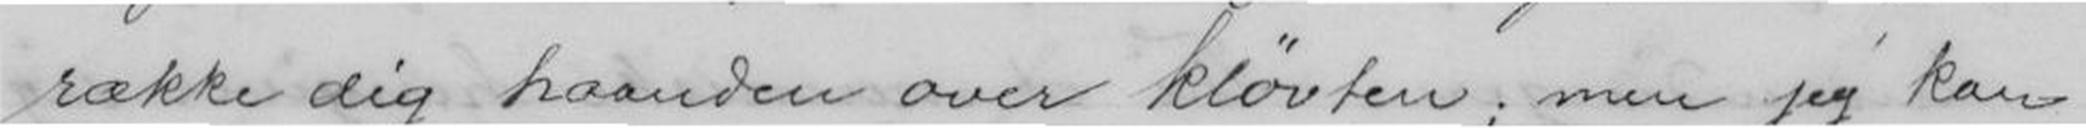række dig haanden over kløvten; men jeg kan
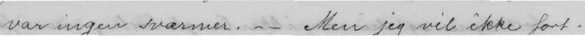var ingen sværmer. - - Men jeg vil ikke fort-
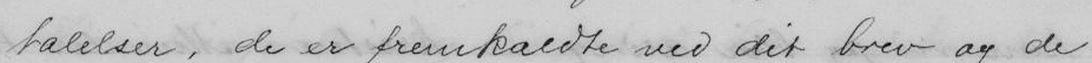talelser, de er fremkaldte ved det brev og de
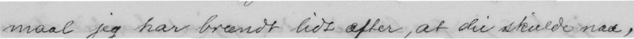maal jeg har brændt lidt æfter, at du skulde naa,
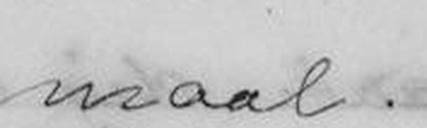maal.
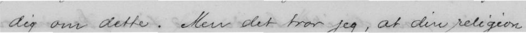dig om dette. Men det tror jeg, at din religion
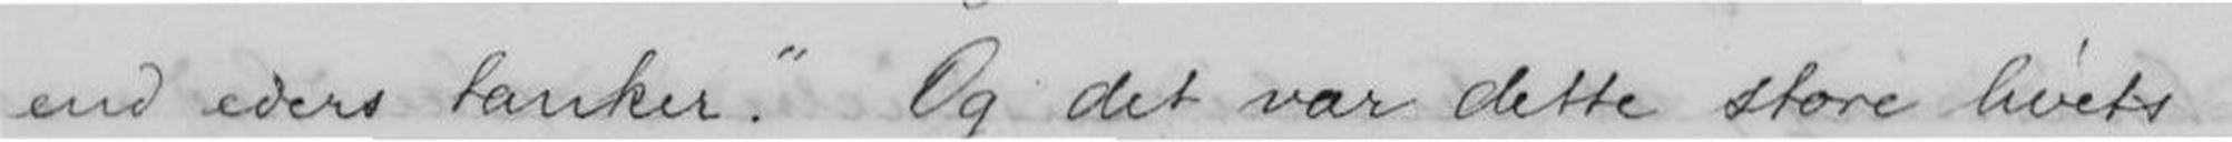end eders tanker. Og det var dette store livets
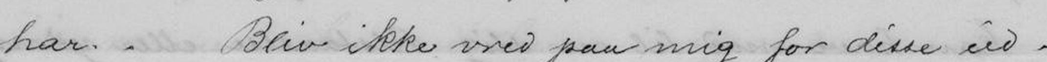har. Bliv ikke vred paa mig for disse ud-
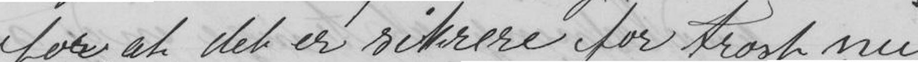for at det er sikrere for Frost nu
